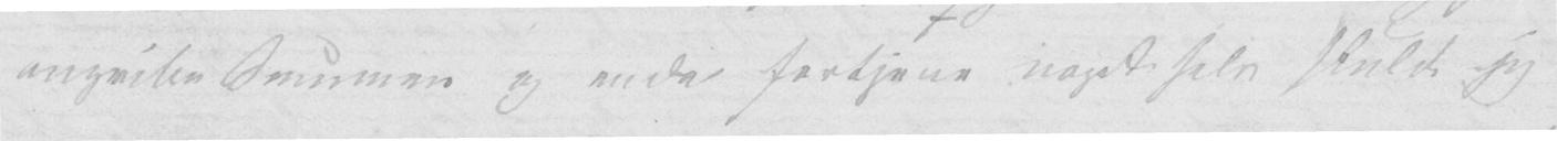angribe Summen og enda fortjene noget selv skulde jeg
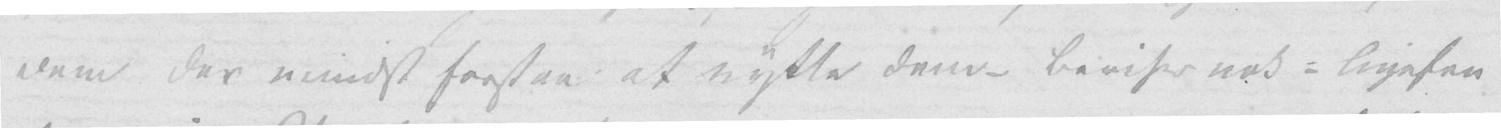dem der mindst forstaa at nytte dem – Beviser nok: ligefra
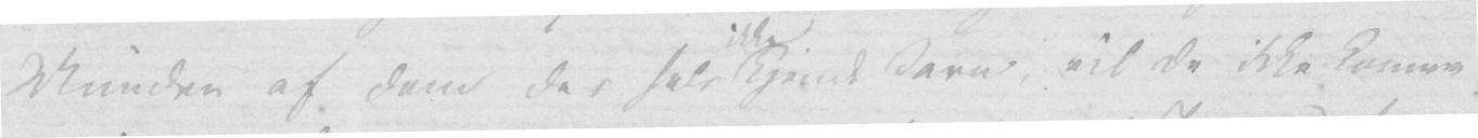Munden af dem der selv kjende Savn, vil De ikke komme
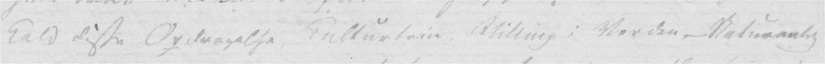kald disse Opdragelse, Kulturtrin, Stilling i Verden – Naturanlæg
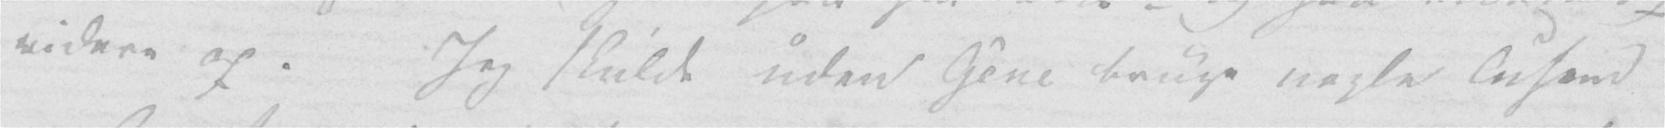videre op. Jeg skulde uden Gêne bruge nogle Tusend
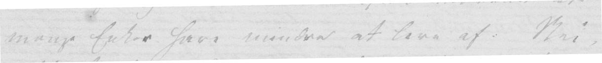mange Enker have mindre at leve af: Nei,
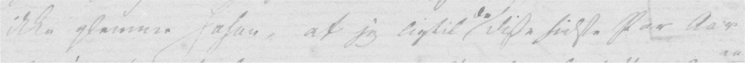ikke glemme Johan, at jeg ligetil de disse sidste Par Aar
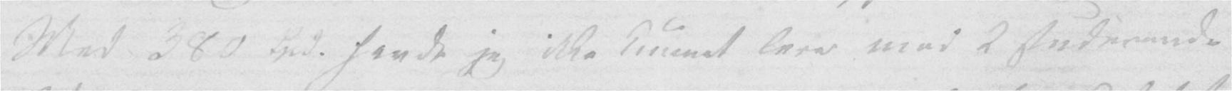Med 380 Spd. havde jeg ikke kunnet leve med 2 studerende
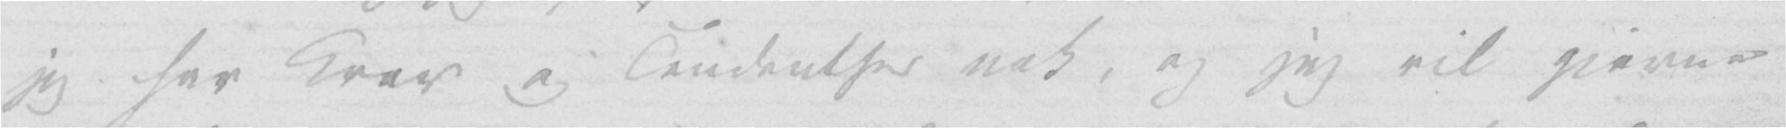jeg har Krav og Tendentser nok, og jeg vil gjerne
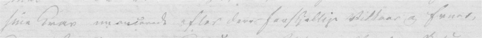sine Krav nuancerede efter deres forskjellige Vilkaar og Evner,
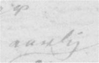aarlig
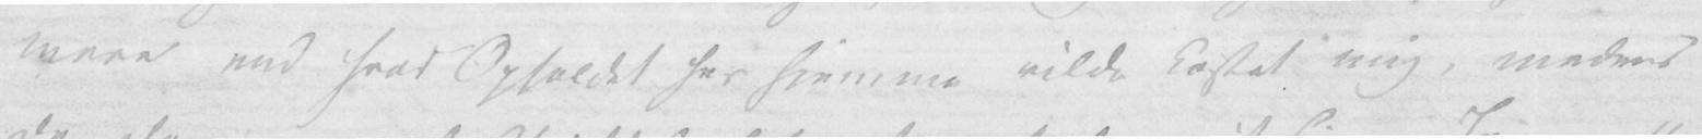mere end hvad Opholdet her hjemme vilde kostet mig, medens
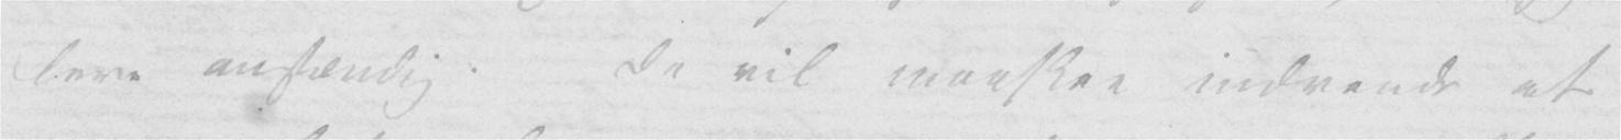leve anstændig. De vil maaskee indvende at
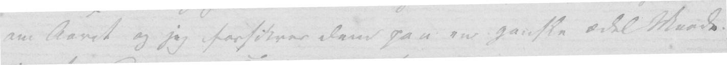om Aaret og jeg forsikrer Dem paa en ganske ædel Maade.
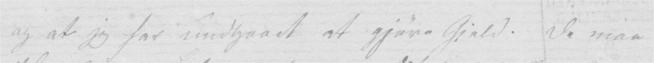og at jeg har undgaaet at gjøre Gjeld. De maa
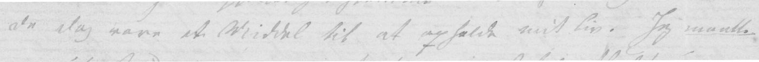de dog vare et Middel til at opholde mit Liv. Jeg maatte
